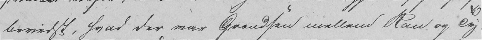bevidst, hvad der var Grændsen mellem Ran og Ty-
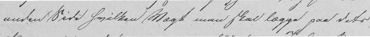anden Side hvilken Vægt man skal lægge paa dets
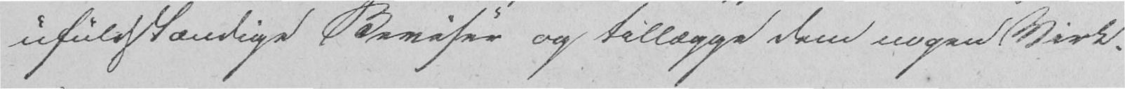ufuldstændige Beviser og tillægge dem nogen Virk-
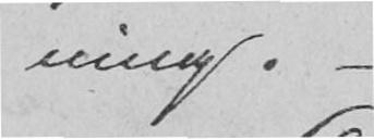ning. -
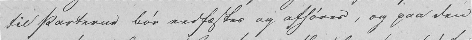til Parterne bør nedfæstes og afhøres, og paa den
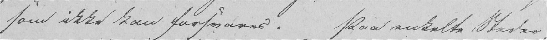som ikke kan forsvares. Paa enkelte Steder,
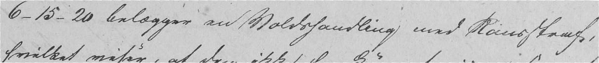6-15-20 belægger en Voldshandling med Ransstraf,
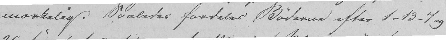mærkelig. Saaledes fordeles Bøderne efter 1-13-7 og
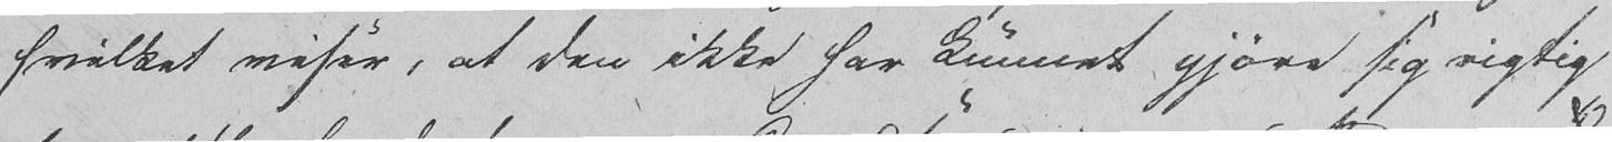hvilket viser, at den ikke har kunnet gjøre sig rigtig
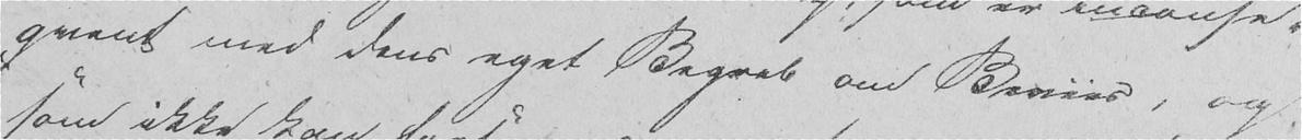qvent med dens eget Begreb om Beviis, og
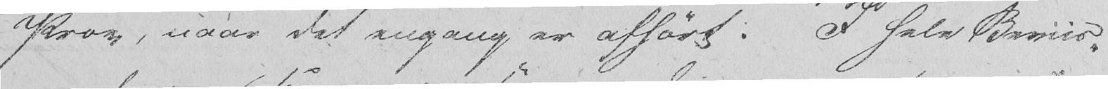Prov, naar det engang er afhørt. I hele Beviis-
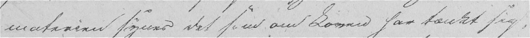materien synes det som om Loven har tænkt sig
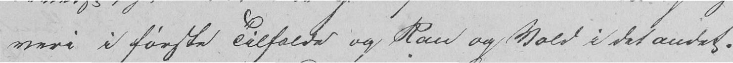veri i første Tilfælde og Ran og Vold i det andet.
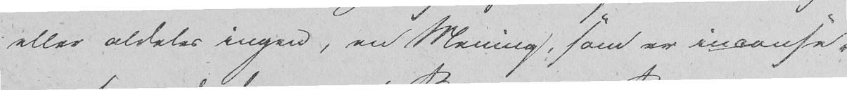eller aldeles ingen, en Mening, som er inconse-
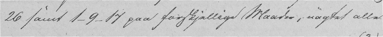26 samt 1-9-17 paa forskjellige Maader, uagtet alle
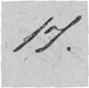17.
